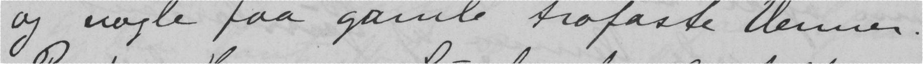og nogle faa gamle trofaste venner.
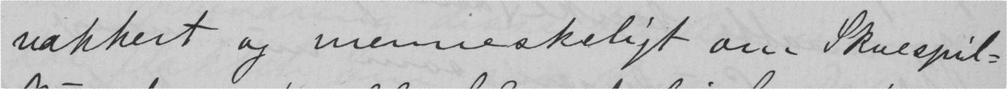vakkert og menneskeligt om Skuespil-
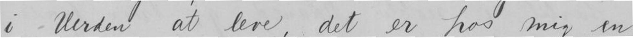i Verden at lere, det er hos mig en
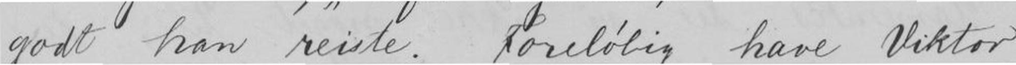godt han reiste. Foreløbig have Viktor
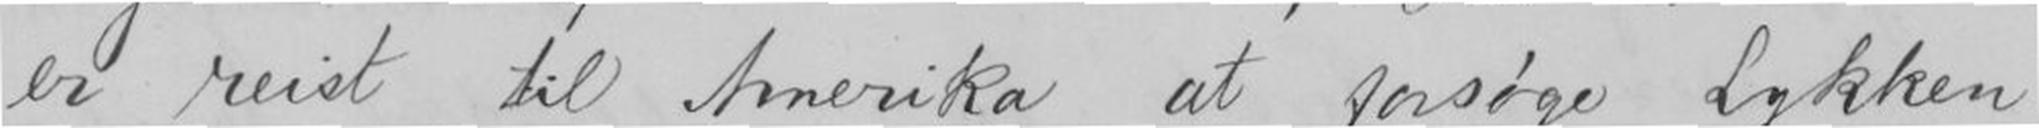er reist til Amerika at forsøge Lykken
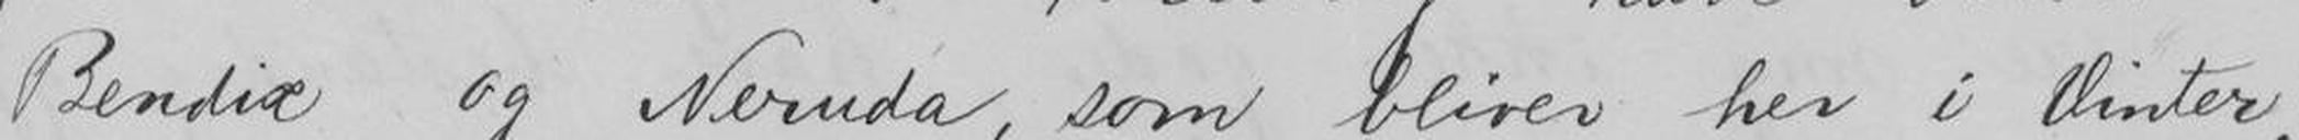Bendix og Neruda, som bliver her i Vinter,
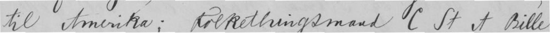til Amerika; Folkethingsmand C St A Bille
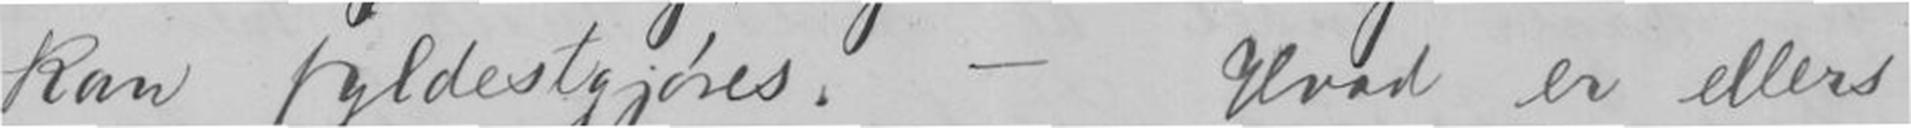kan fyldestgjøres. - Hvad er ellers
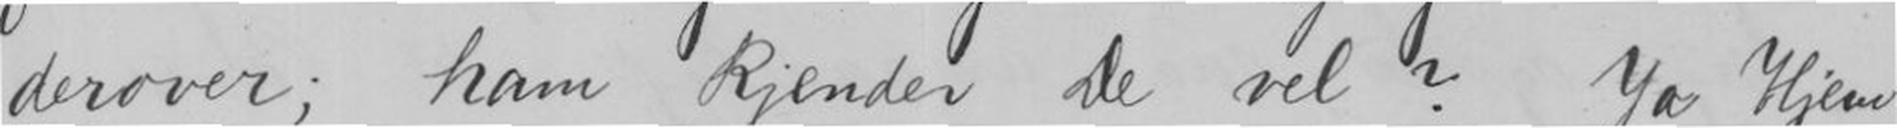derover; ham kjender De vel ? Ja Hjem-
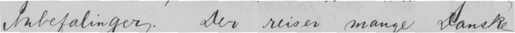Anbefalinger. Der reiser mange Danske
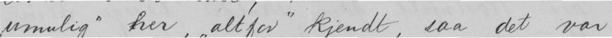umulig her, altfor kjendt, saa det var
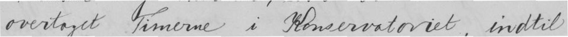overtaget Timerne i Konservatoriet, indtil
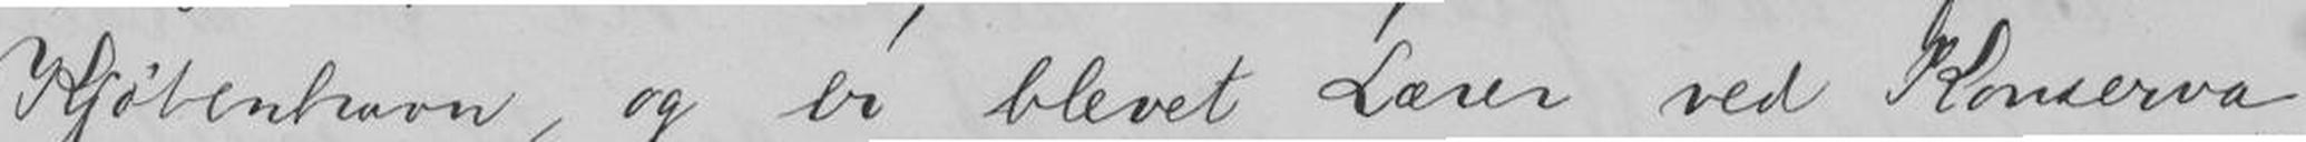Kjøbenhavn, og er blevet Lærer ved Konserva-
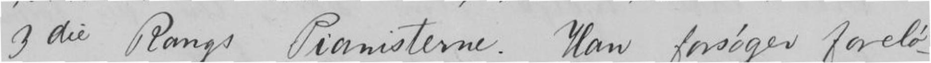3die Rangs Pianisterne. Han forsøger forelø-
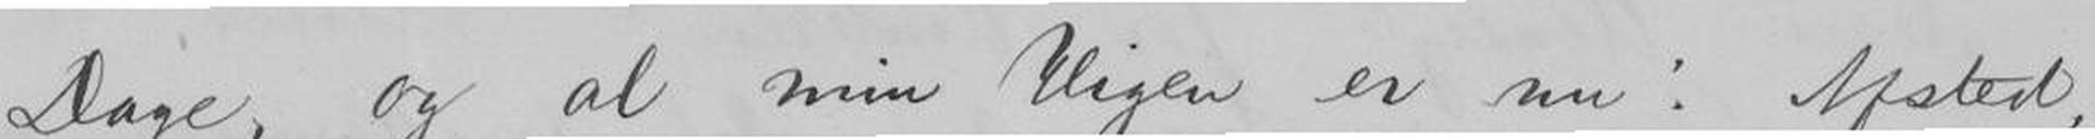Dage, og al min Higen er nu: Afsted,
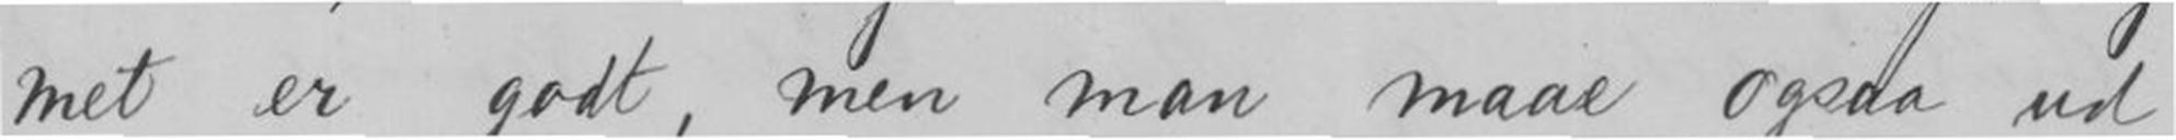met er godt, men man maae ogsaa ud
